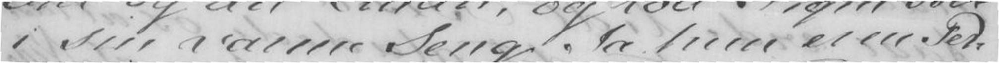i sin varme Seng. Ja hun er en Per-
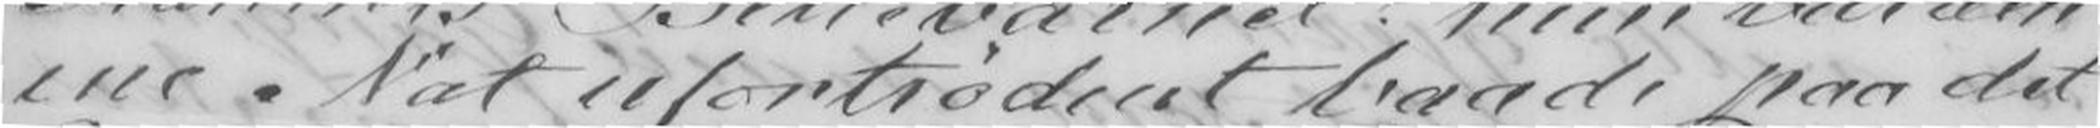ene Nat ufortrødent baade paa det
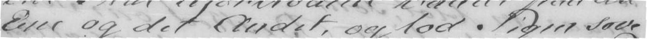Ene og det Andet, og lod Pigen sove
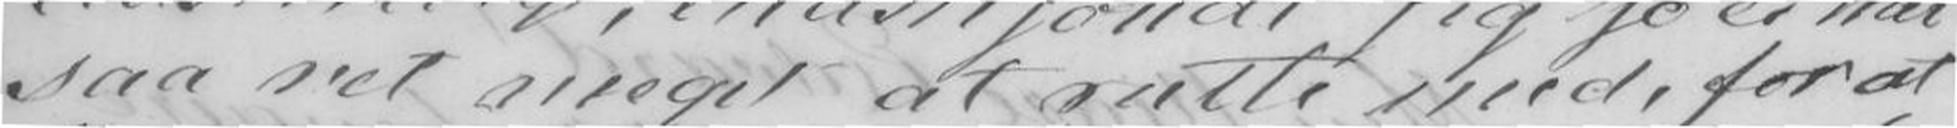saa ret meget at rutte med, for at
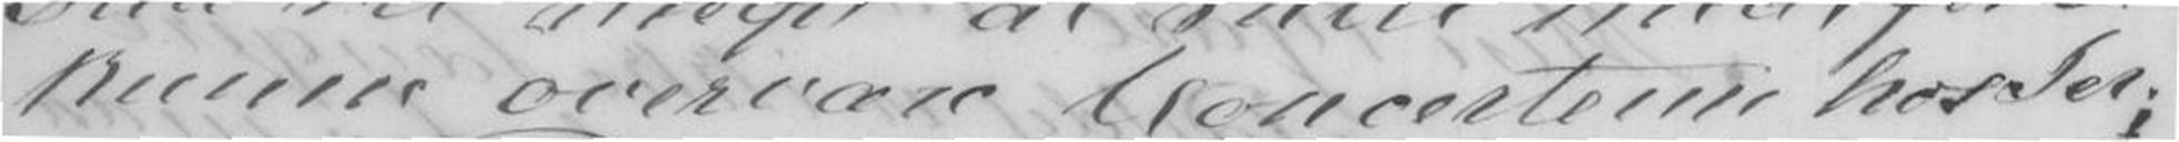kunne overvære Concerterne hos Jer;
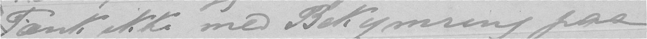Tænk ikke med Bekymring paa
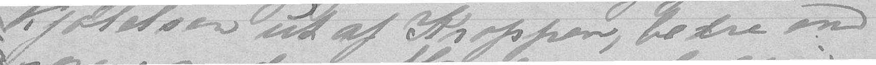kjølelsen ut af Kroppen, bedre end
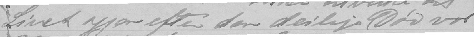Livet igjen efter den deilige Død vor
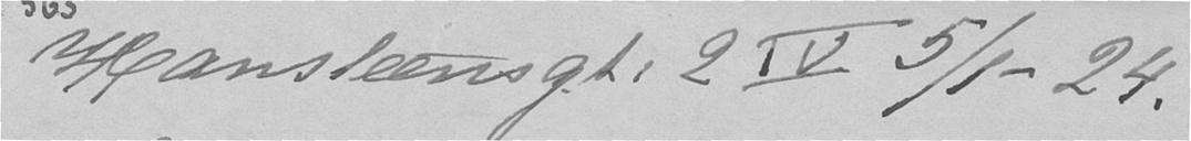Hansteensgt. 2 IV 5/1- 24.
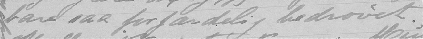bare saa forfærdelig bedrøvet.
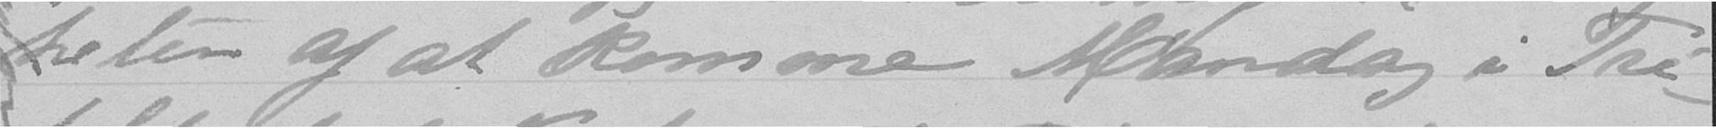heten af at komme Mandag i Tre-
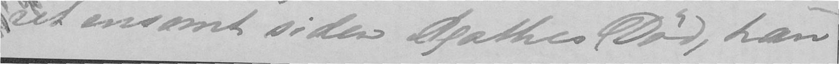ret ensomt siden Agathes Død, han
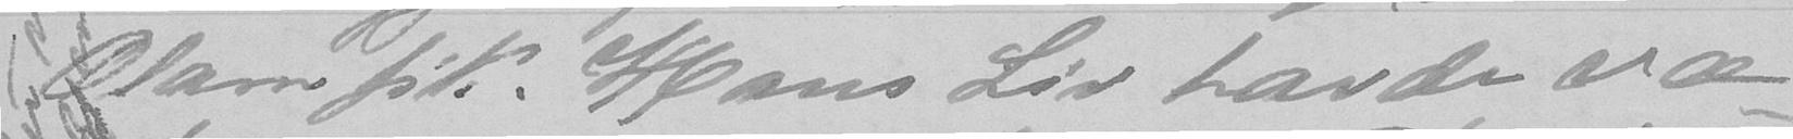Olaus fik. Hans Liv havde væ-
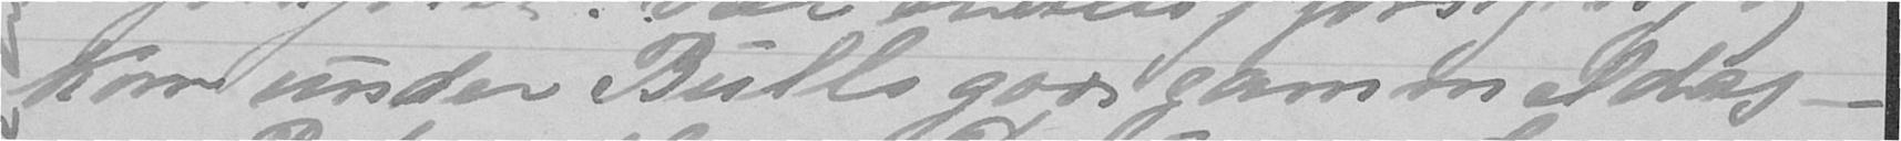kom under Bulls gode gammeldag-
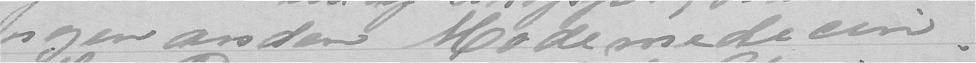nogen anden Modemedicin.
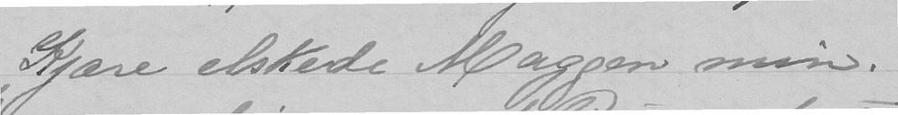Kjære elskede Maggen min.
